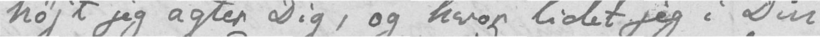højt jeg agter Dig, og hvor lidet jeg i Din
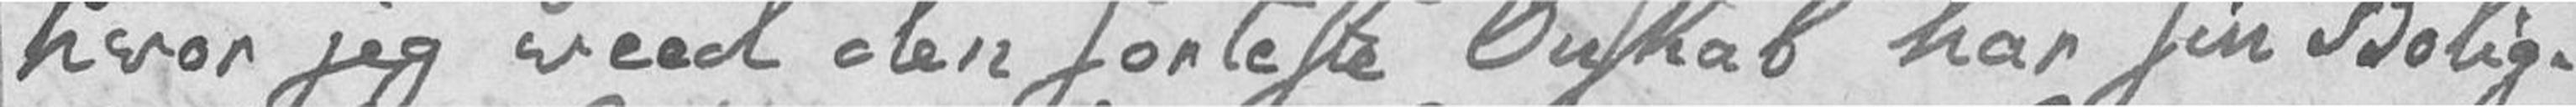hvor jeg veed den sorteste Onskab har sin Bolig.
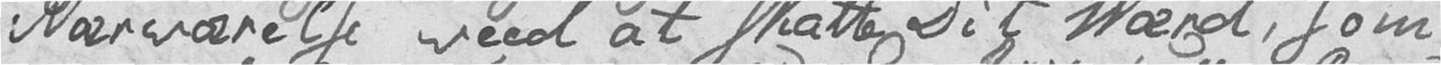Nærværelse veed at skatte Dit Værd, som
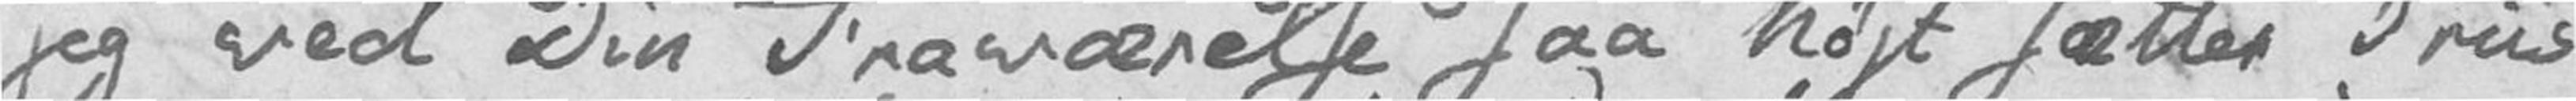jeg ved Din Fraværelse saa højt sætter Priis
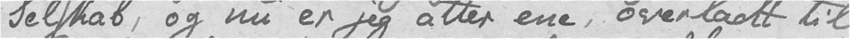Selskab, og nu er jeg atter ene, overladt til
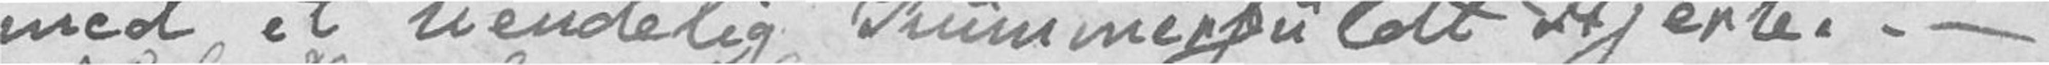med et uendelig Kummerfuldt Hjerte. – –
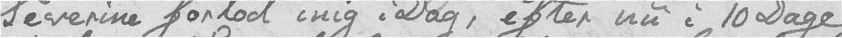Severine forlod mig i Dag, efter nu i 10 Dage
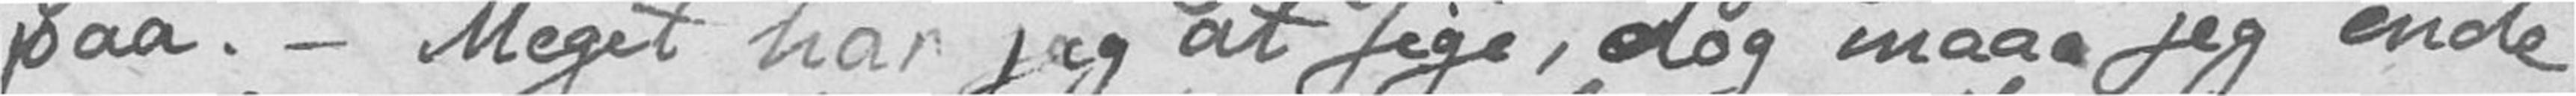paa. – Meget har jeg at sige, dog maae jeg ende
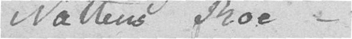Nattens Roe –
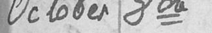October 8de
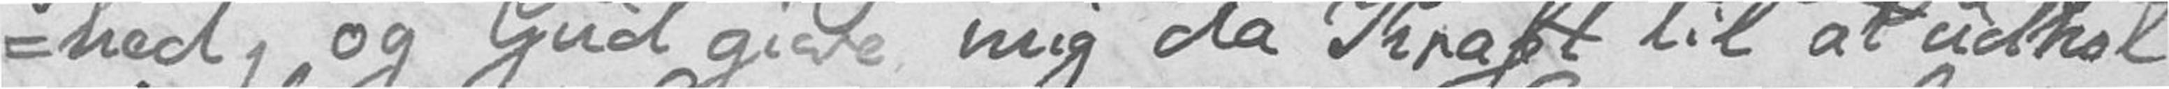-hed, og Gud give mig da Kraft til at udhol
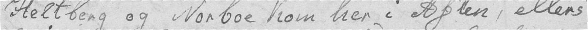Heltberg og Norboe kom her i Aften, ellers
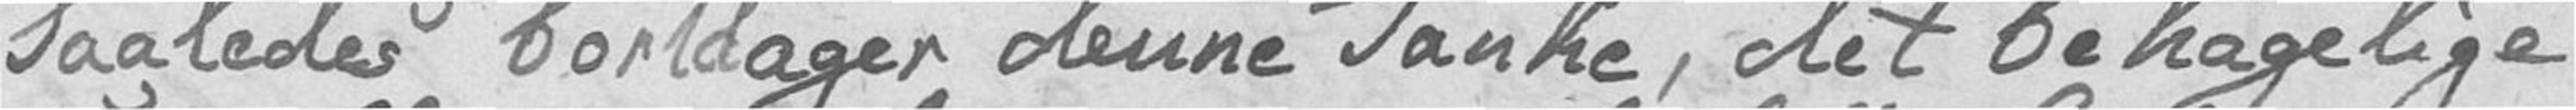Saaledes borttager denne Tanke, det behagelige
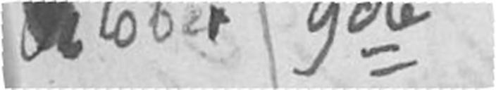October 9de
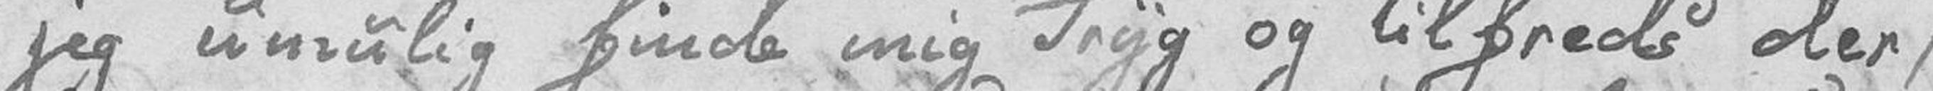jeg umulig finde mig Tryg og tilfreds der,
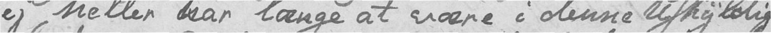ej heller har længe at være i denne Uskyldig
